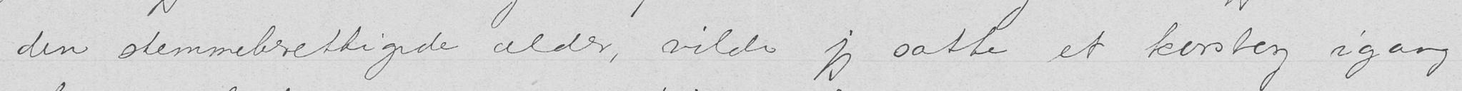den stemmeberettigede alder, vilde jeg sætte et korstog igang
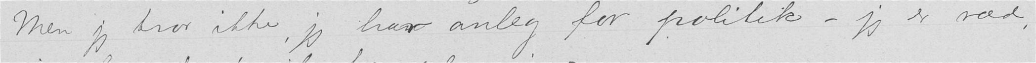Men jeg tror ikke, jeg har anleg for politik - jeg er ræd,
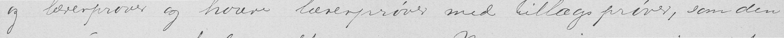og lærerprøver og høiere lærerprøver med tillægsprøver, som den
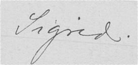Sigrid.
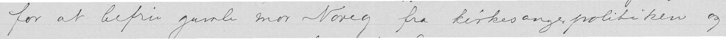for at befri gamle mor Noreg fra kirkesangerpolitiken og
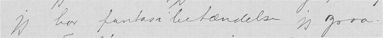jeg har fantasibetændelse jeg ogsaa.
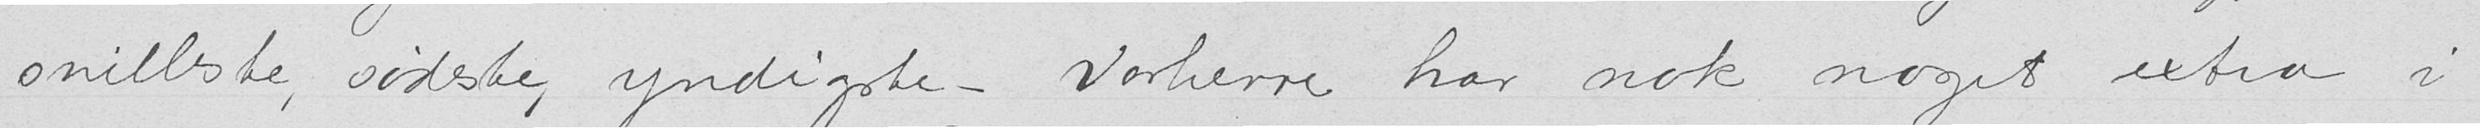snilleste, sødeste, yndigste - Vorherre har nok noget extra i
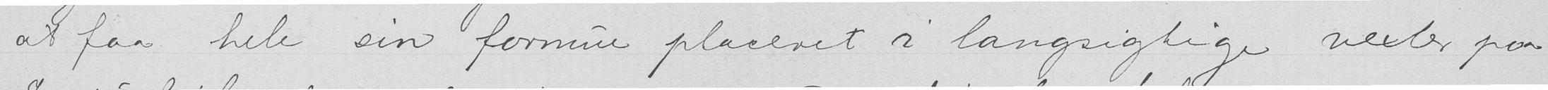at faa hele sin formue placeret i langsigtige vexler paa
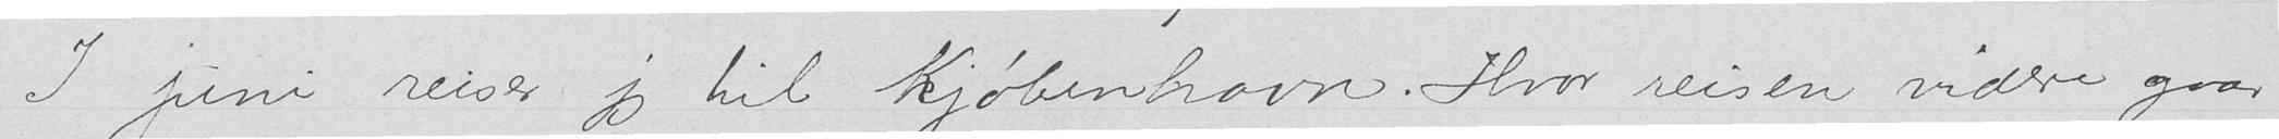I juni reiser jeg til Kjøbenhavn. Hvor reisen videre gaar
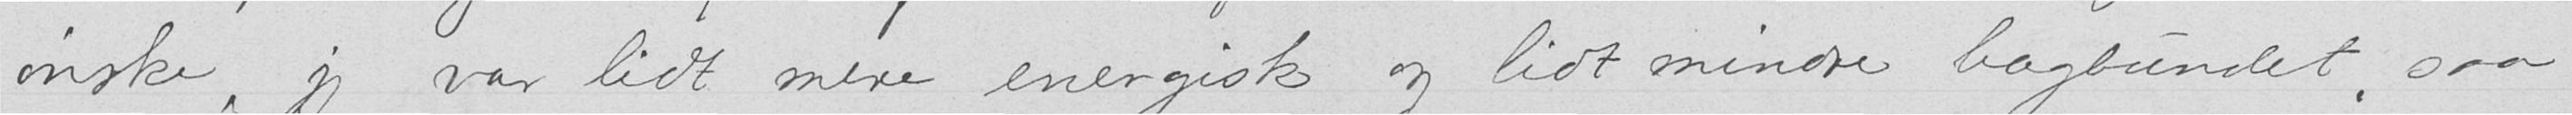ønske, jeg var lidt mere energisk og lidt mindre bagbundet, saa
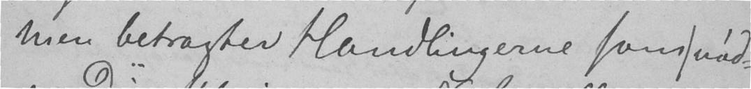men betragter Handlingerne som nød-
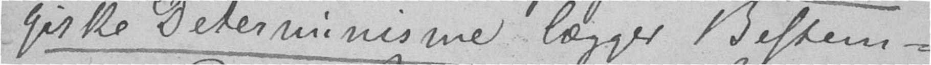giske Determinisme lægger Bestem-
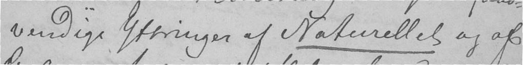vendige Ytringer af Naturell el og af
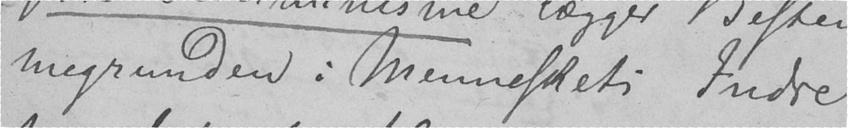megrunden i Menneskets Indre
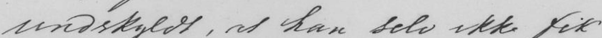undskyldt, at han selv ikke fik
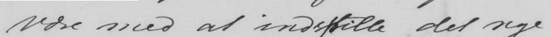være med at indstille det nye
